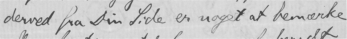derved fra Din Side er noget at bemærke
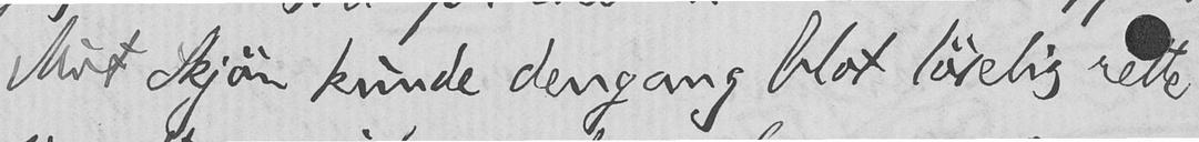Mit Skjøn kunde den gang blot løselig rette
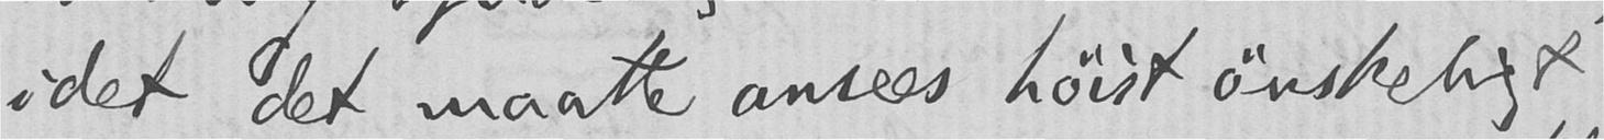idet det maatte ansees høist ønskeligt
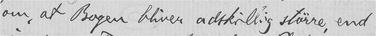om, at Bogen bliver adskillig større end
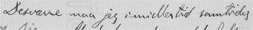Desværre maa jeg imidlertid samtidig
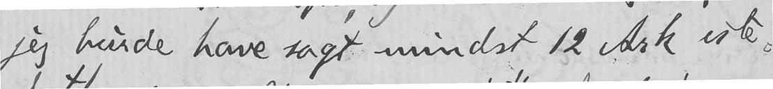jeg burde have sagt mindst 12 Ark iste-
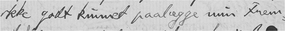ikke godt kunnet paalægge min Frem-
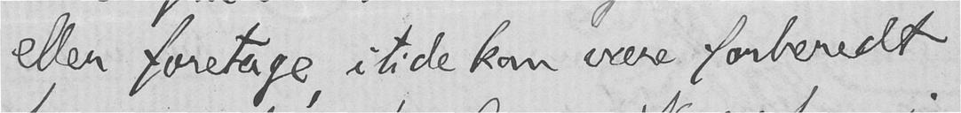eller foretage, itide kan være forbededt
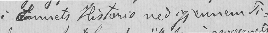i Emnets Historie ned igjennem Ti-
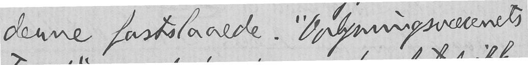derne fastslaaede. "Oplysningsvæsenets
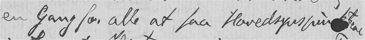en Gang for alle at faa Hovedsynspunkterne
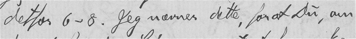detfor 6-8. Jeg nævner dette, forat Du, om
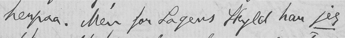herpaa. Men for Sagens Skyld har jeg
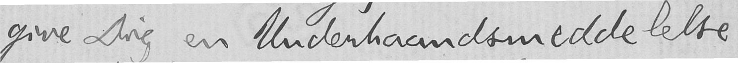give Dig en Underhaandsmeddelelse
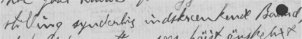stilling synderlig indskrænkende Baand,
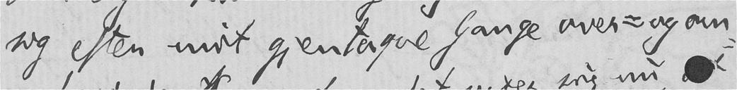sig efter mit gjentagne Gange over- og om-
